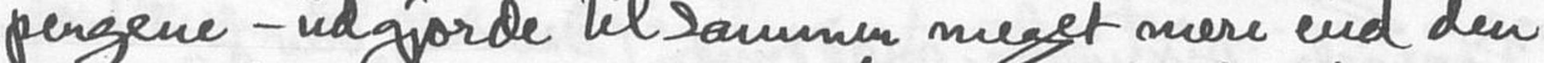pengene - udgjorde tilsammen meget mere end den
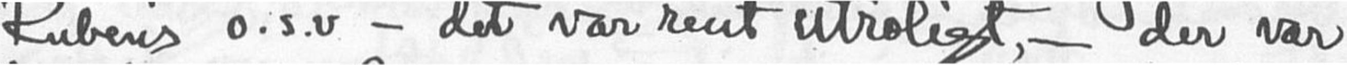Rubens o.s.v. - det var rent utroligt, - der var
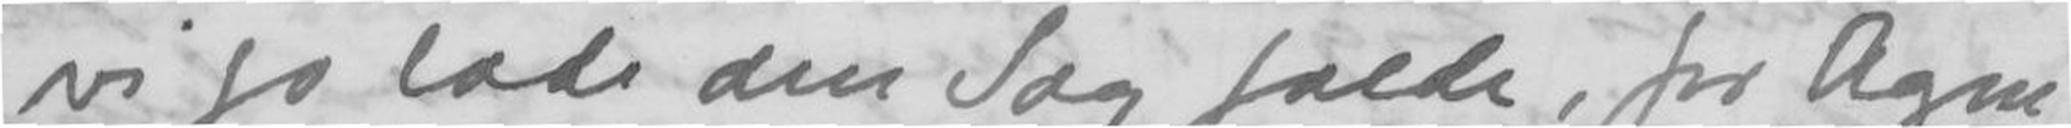vi jo lade den Sag falde, for Agen-
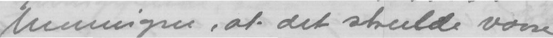meningen at det skulde vaere
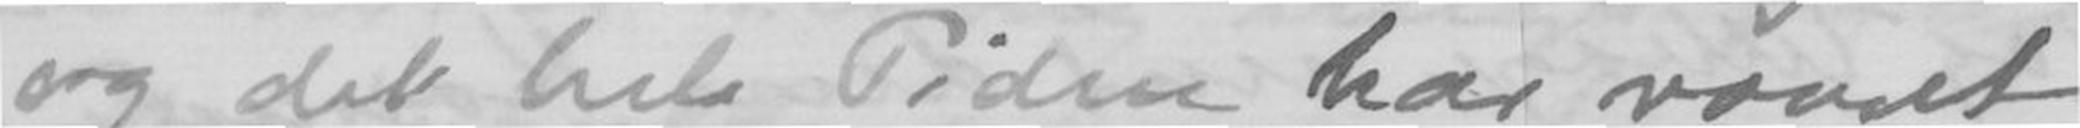og det hele Tiden har vaeret
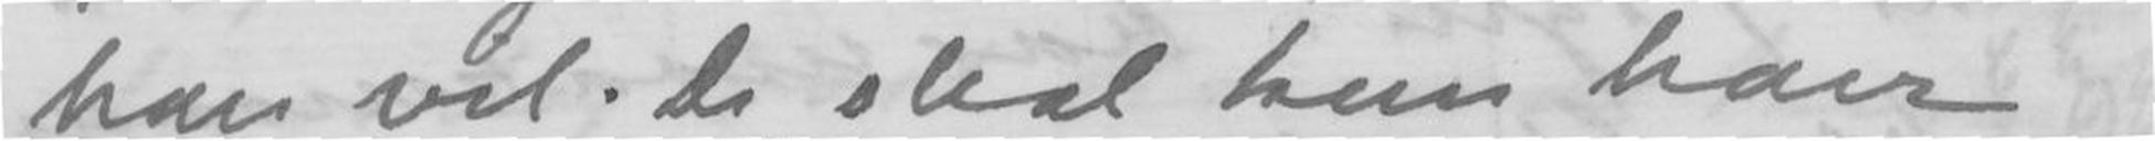han vil. De skal kun have
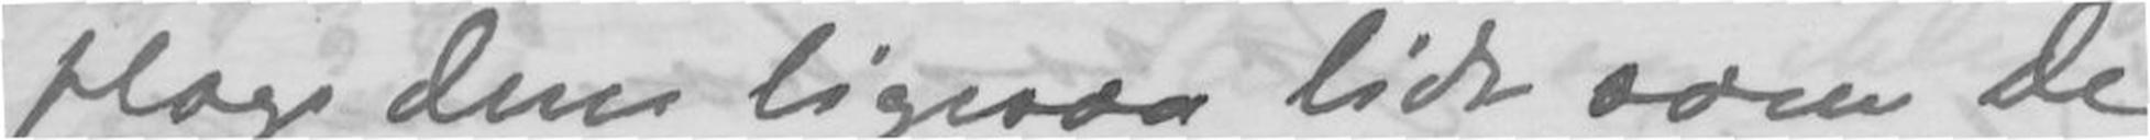plage dem ligesaa lidt som De
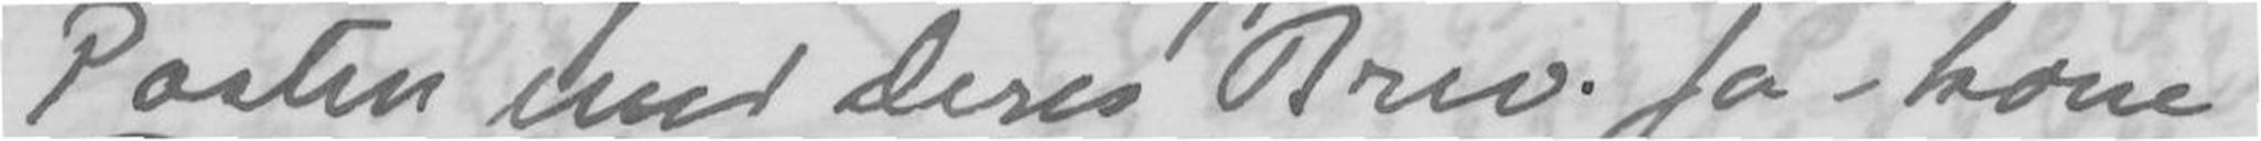Posten med Deres Brev. Ja - kaere
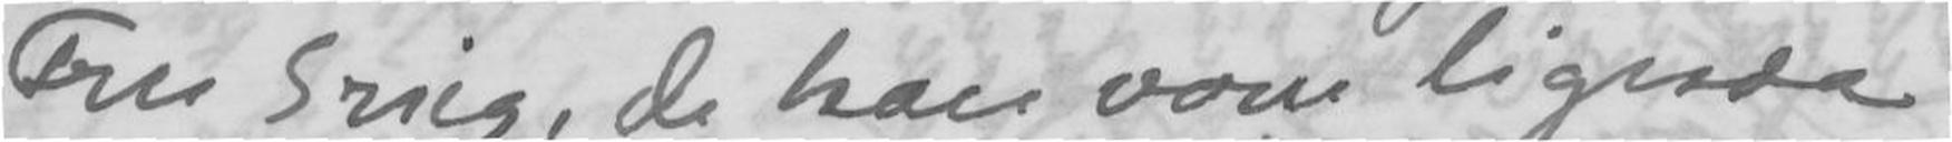Fru Grieg, de kan vaere ligesaa
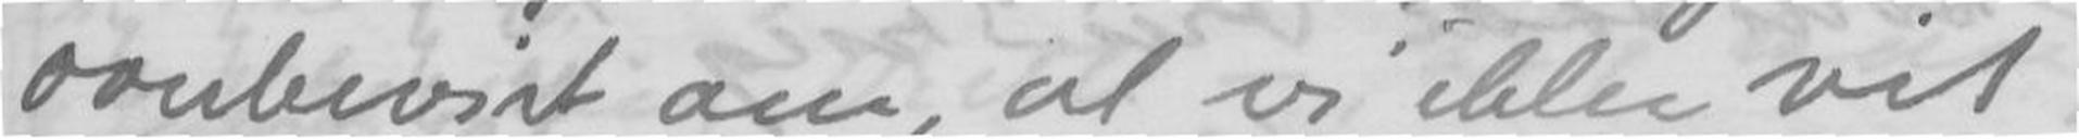overbevist om, at vi ikke vil
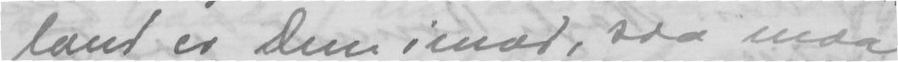land er Dem imod, saa maa
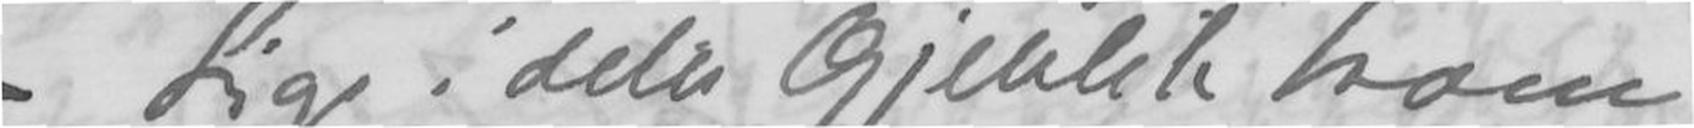- Lige i dette Øjeblik kom
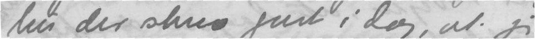ten der skrev just i dag, at jeg
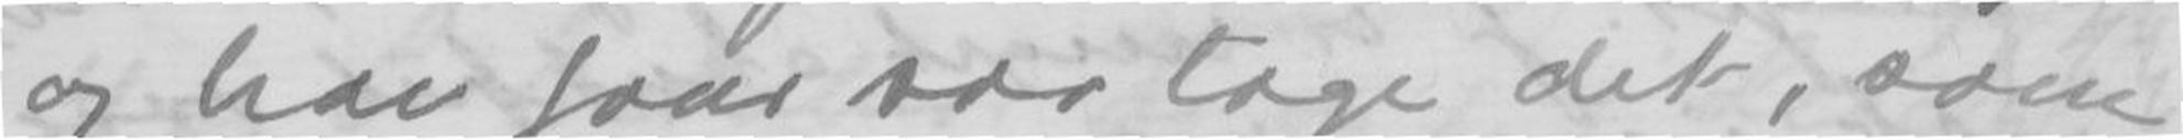og han faar saa tage det, som
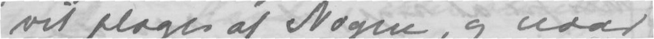vil plages ag Nogen, og naar
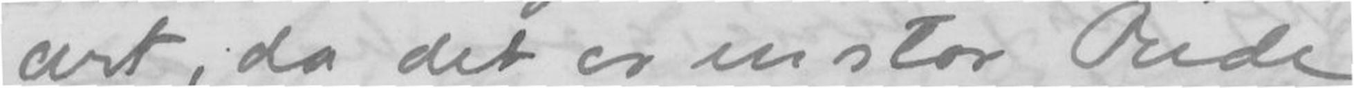cert, da det er en stor Rede
